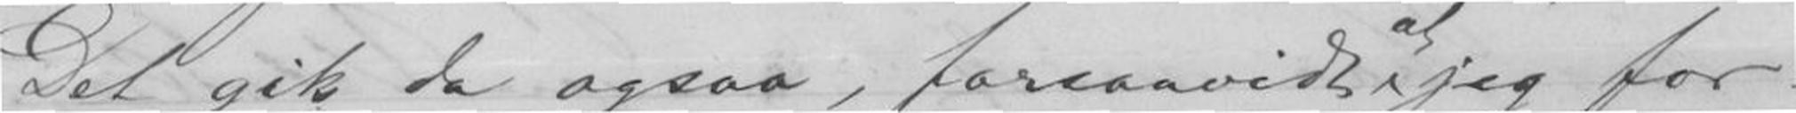Det gik da ogsaa, forsaavidt at jeg for-
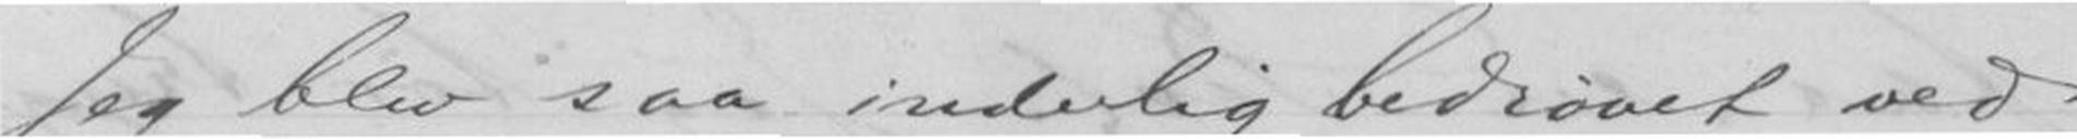Jeg blev saa inderlig bedrøvet ved
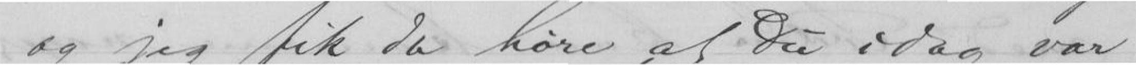og jeg fik da høre, at Du idag var
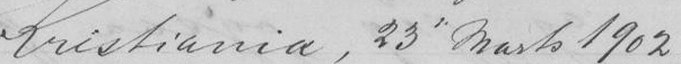Kristiania, 23 Marts 1902.
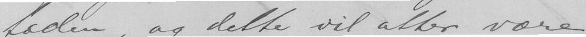tiden, og dette vil atter være
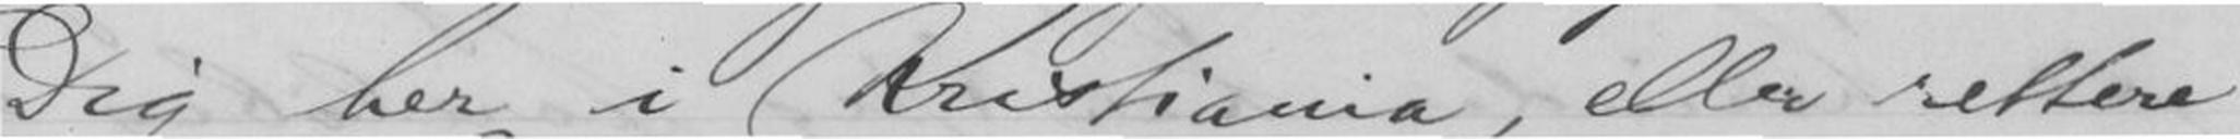Dig her i Kristiania, eller rettere
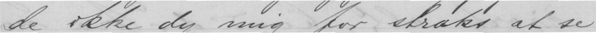de ikke dy mig for straks at se
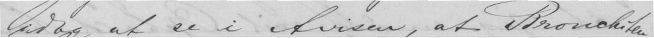idag at se i Avisen, at Bronchiten
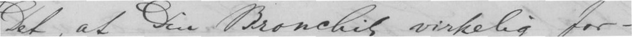Det, at Din Bronchit virkelig for-
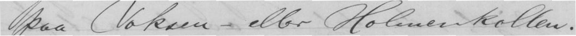paa Voksen- eller Holmenkollen.
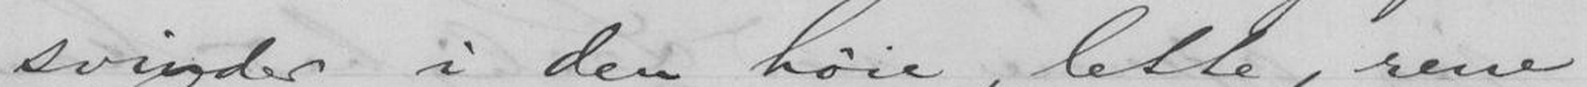svinder i den høie, lette, rene
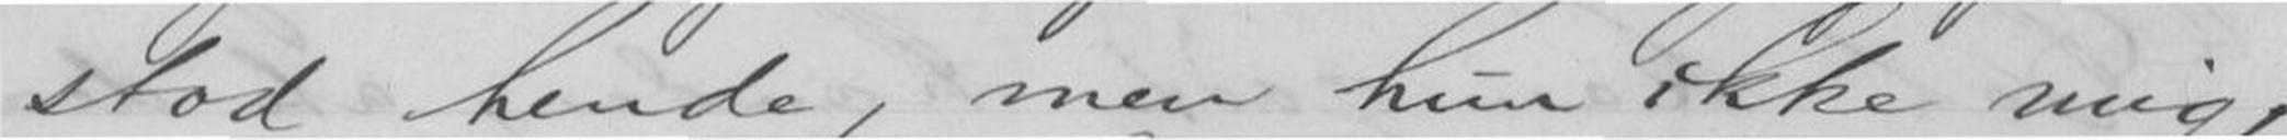stod hende, men hun ikke mig,
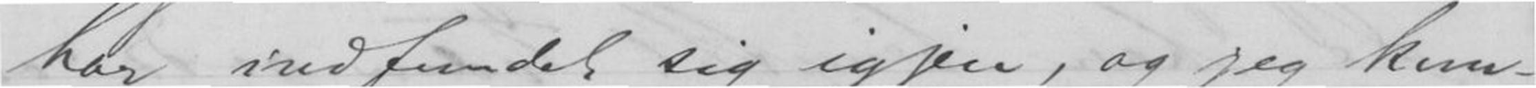har indfundet sig igjen, og jeg kun-
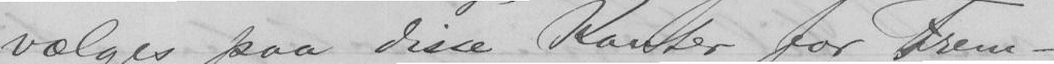vælges paa disse Kanter for Frem-
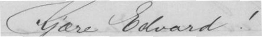Kjære Edvard!
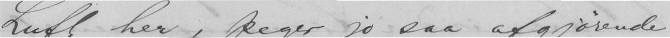Luft her, peger jo saa afgjørende
